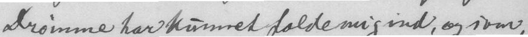Drømme har kunnet falde mig ind, og som,
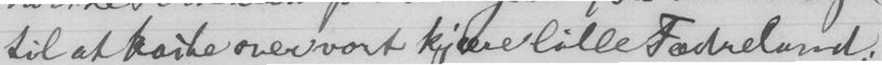til at kaste over vort kjære lille Fædreland,
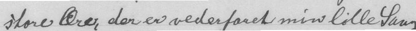store Ære, der er vederfaret min lille Sang
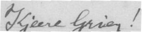Kjære Grieg!
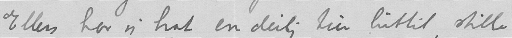Ellers har vi hat en deilig tur hittil, stille
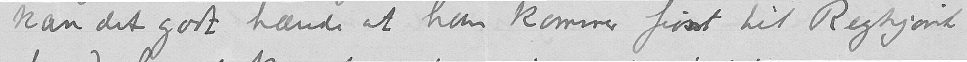kan det godt hænde at han kommer først til Reykjavik
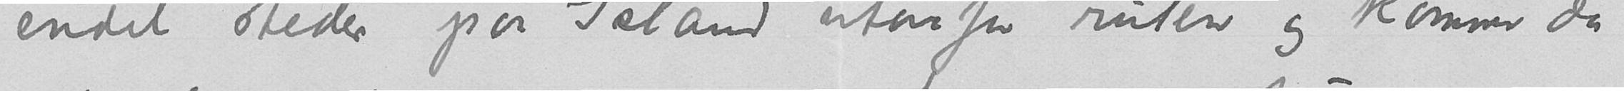endel steder paa Island utenfor ruten og kommer da
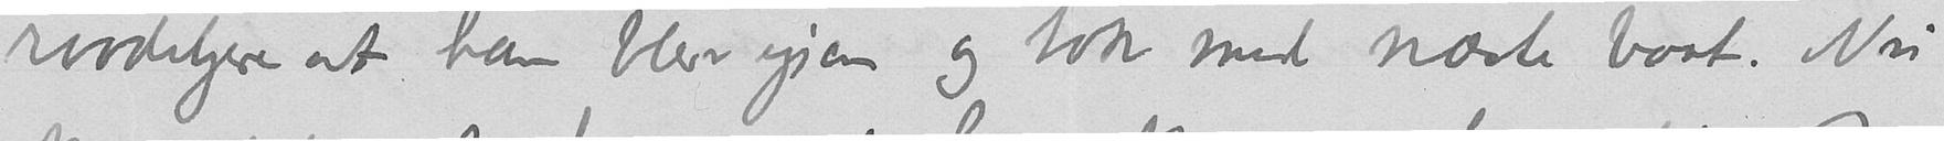raadeligere at han blev igjen og tok med næste baat. Nu
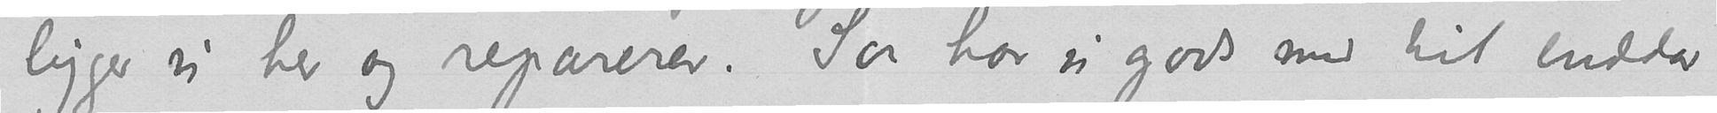ligger vi her og reparerer. Saa har vi gods med til endda
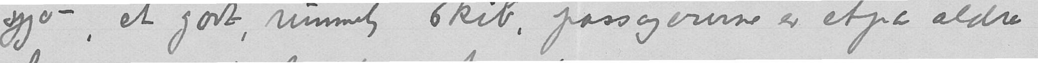sjø -, et godt, rummelig skib, passagererne er etpar eldre
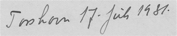Torshavn 17. Juli 1931.
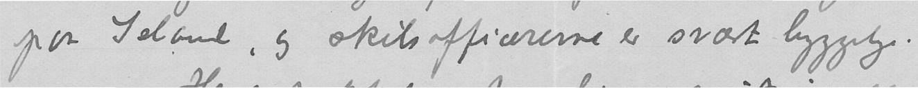paa Island, og skibsofficererne er svært hyggelige.
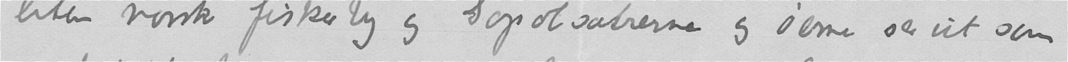liten norsk fiskerby og Gopolsætrerne og øerne ser ut som
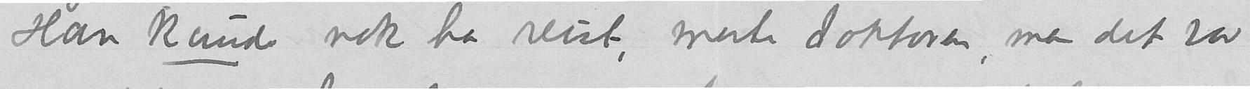Han kunde nok ha reist, mente doktoren, men det var
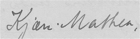Kjære Mathea,
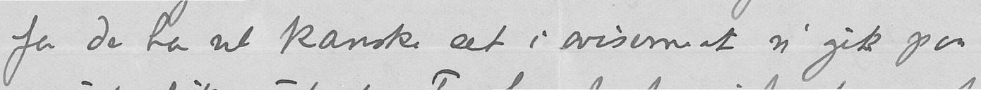for De har vel kanske set i aviserne at vi gik paa
